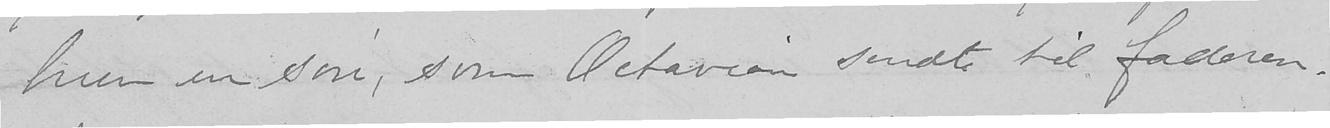hun en søn, som Octavian sendte til faderen.
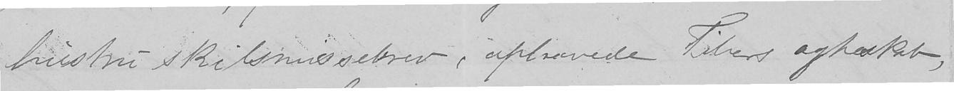hustru skilsmissebrev, ophævede Tibers ægteskab,
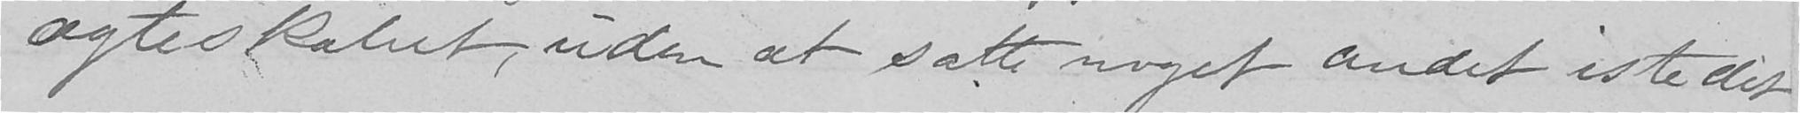ægteskabet, uden at sætte noget andet istedet,
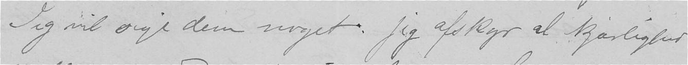Jeg vil sige dem noget: jeg afskyr al Kjærlighed
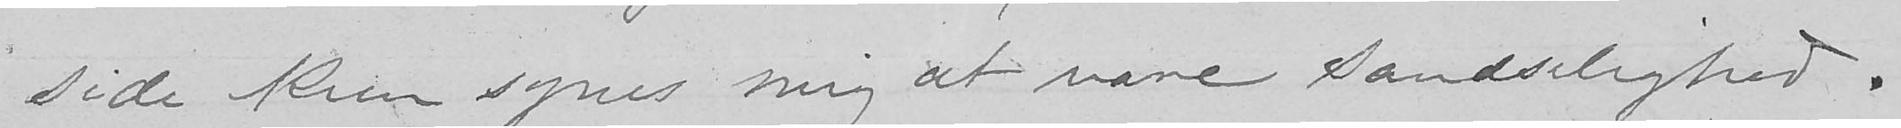side kun synes mig at være sandselighed.
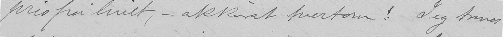pris på livet, - akkurat tvertom! Jeg trives
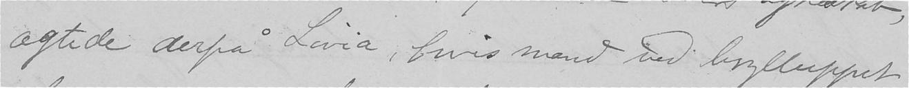ægtede derpå Livia, hvis mand ved brylluppet
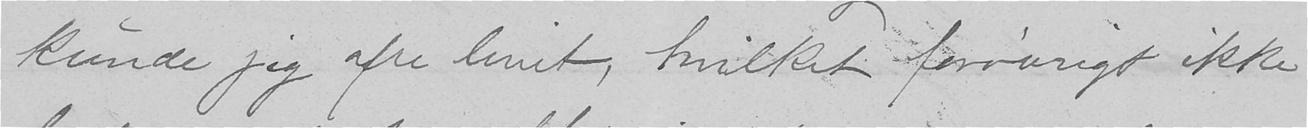kunde jeg ofre livet, hvilket forøvrigt ikke
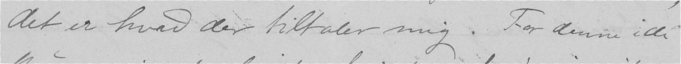det er hvad der tiltaler mig. For denne idé
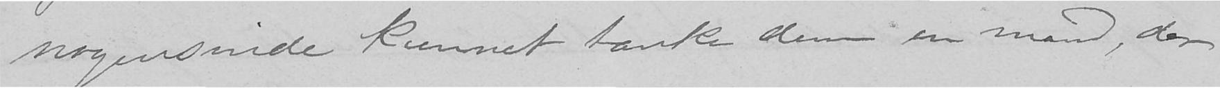nogensinde kunnet tænke dem en mand, der
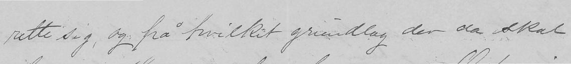rette sig, og på hvilket grundlag der da skal
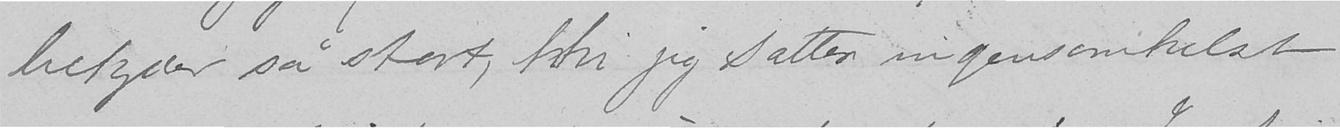betyder så stort, thi jeg sætter ingensomhelst
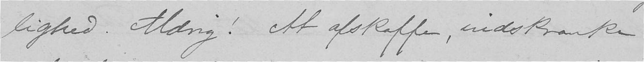lighed. Aldrig! At afskaffe, indskrænke
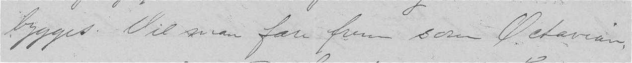bygges. Vil man fare frem som Octavian,
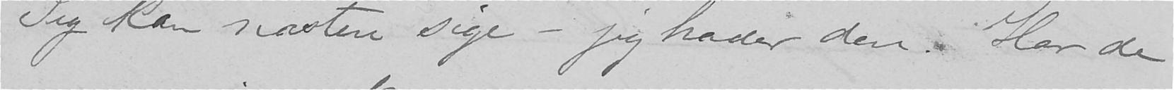Jeg kan næsten sige - jeg hader den. Har de
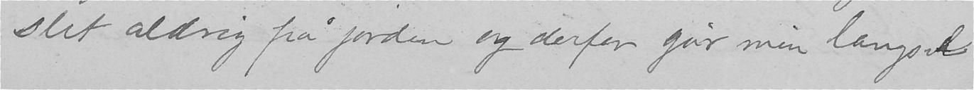slet aldrig på jorden og derfor går min længsel
